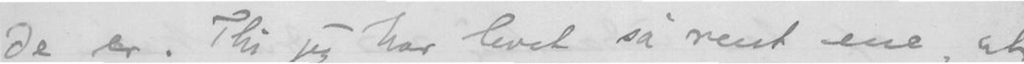de er. Thi jeg har levet så rent ene, at
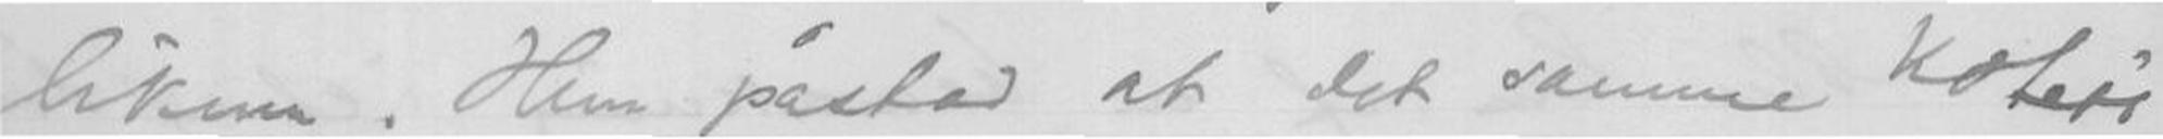likum. Hun påstod at det samme koteri
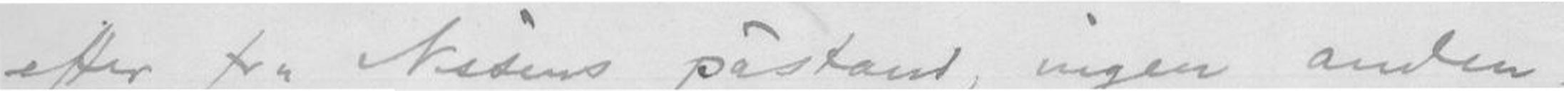efter fr. Nissens påstand, ingen anden,
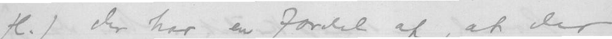fl.) der har en fordel af, at der
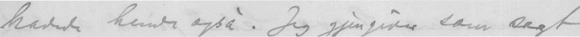hadede hende også. Jeg gjengives som sagt
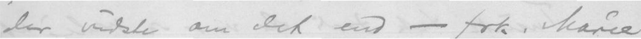der vidste om det end - frk. Marie
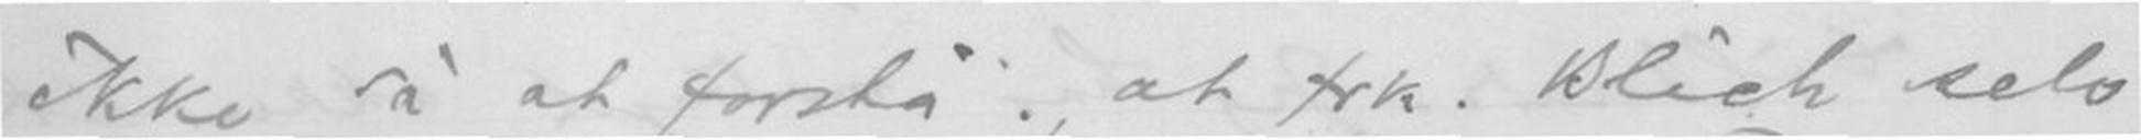ikke så at forstå, at frk. Blich selv
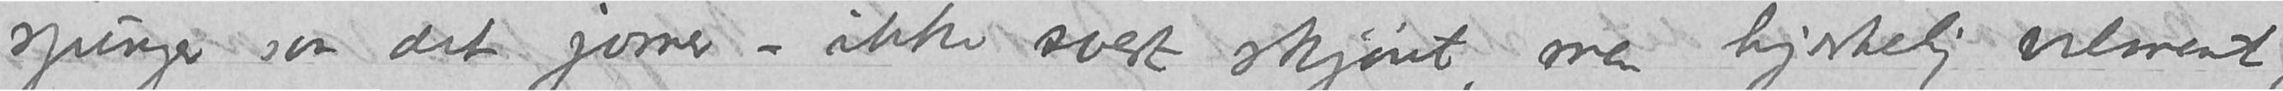sjunger som det jomer – ikke svært skjønt, men hjertelig velment,
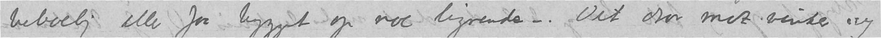beboelig eller faa bygget op noe lignende –. Det drar mot vinter og
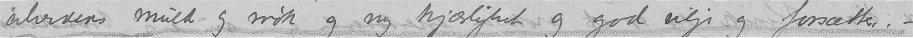alverdens muld og møk og ny kjærlighet og god vilje og forsætter. –
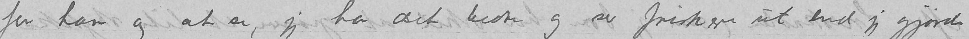for ham og at se, jeg har det bedre og ser friskere ut end jeg gjorde
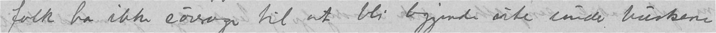folk har ikke courage til at bli liggende ute under buskerne
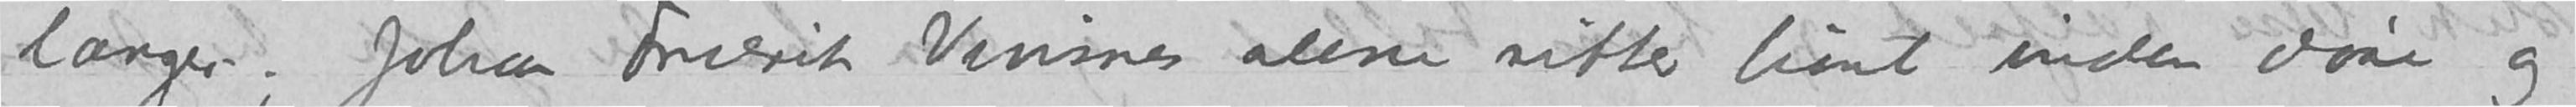længer; Johan Fredrik Vinsnes alene sitter lunt inden døre og
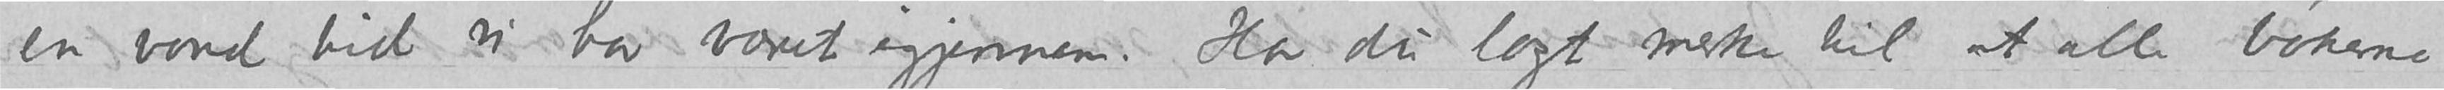en vond tid vi har været igjennem. Har du lagt merke til at alle bøkerne
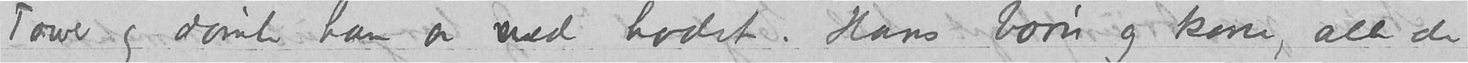Tower og dømte ham av med hodet. Hans børn og kone, alle de
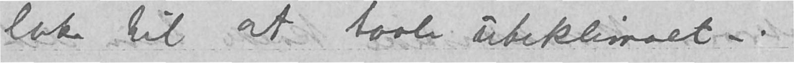later til at taale uteklimaet –.
